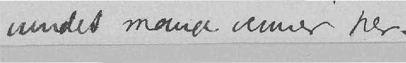vundet mange venner her.
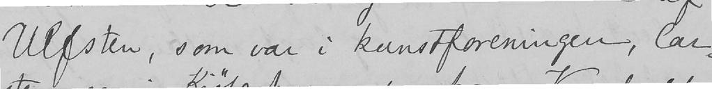Ulfsten, som var i kunstforeningen, Car-
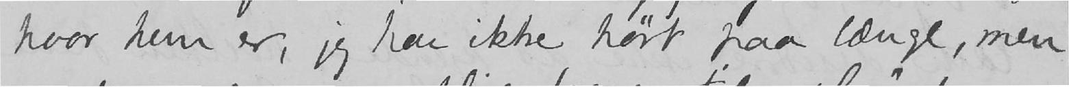hvor hun er, jeg har ikke hørt paa længe, men
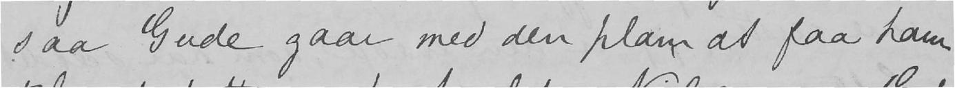saa Gude gaar med den plan at faa ham
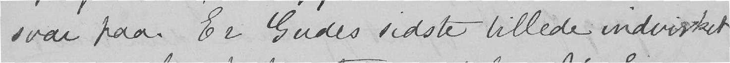svar paa. Er Gudes sidste billede indvirket
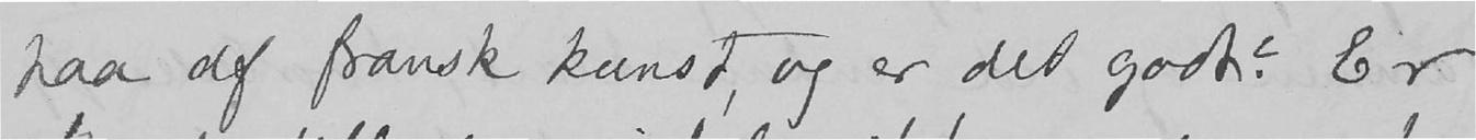paa af fransk kunst, og er det godt? Er
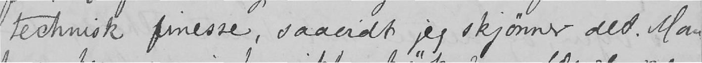technisk finesse, saavidt jeg skjønner det. Mon
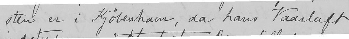sten er i Kjøbenhavn, da hans Vaarluft
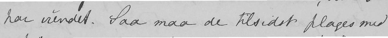har vundet. Saa maa de tilsidst plages med
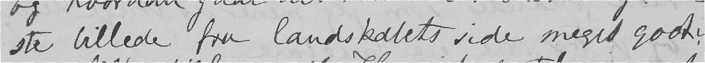ste billede fra landskabets side meget godt?
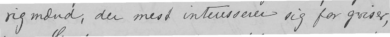rigmænd, der mest interesserer sig for griser,
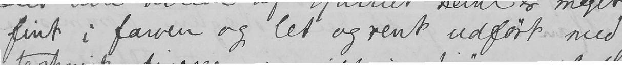fint i farven og let og rent udført med
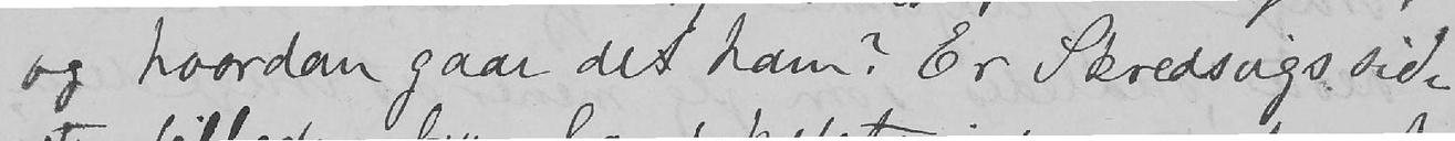og hvordan gaar det ham? Er Skredsvigs sid-
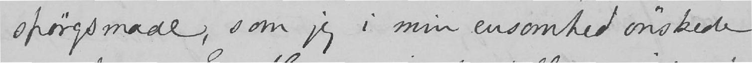spørgsmaal, som jeg i min ensomhed ønskede
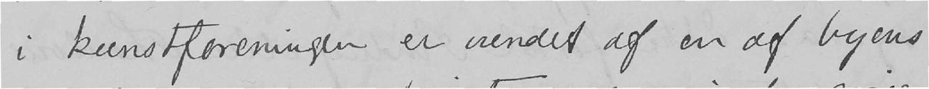i kunstforeningen er vundet af en af byens
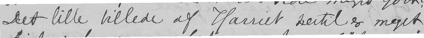Det lille billede af Harriet hertil er meget
